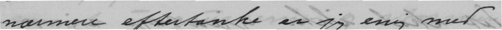nærmere eftertanke er jeg enig med
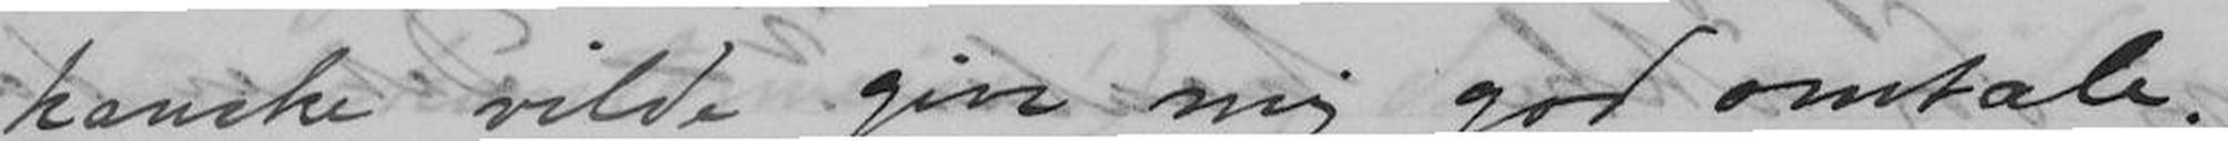kanske vilde give mig god omtale. -
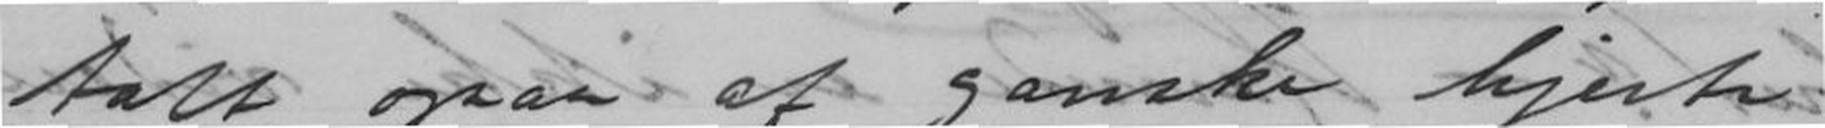talt ogsaa af ganske hjerte
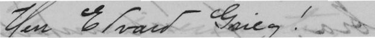Herr Edvard Grieg!
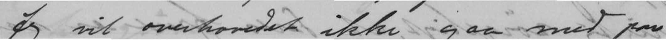Jeg vil overhovedet ikke gaa med paa
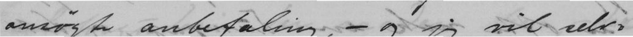omsøgte anbefaling, - og jeg vil selv-
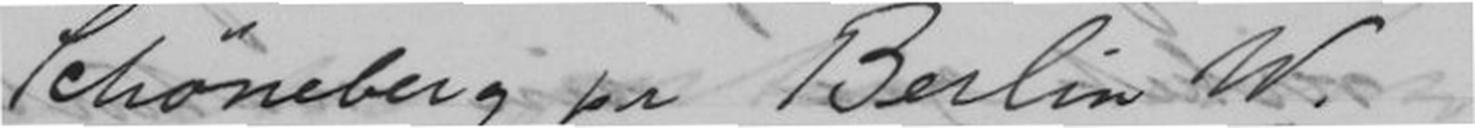Schøneberg pr Berlin W.
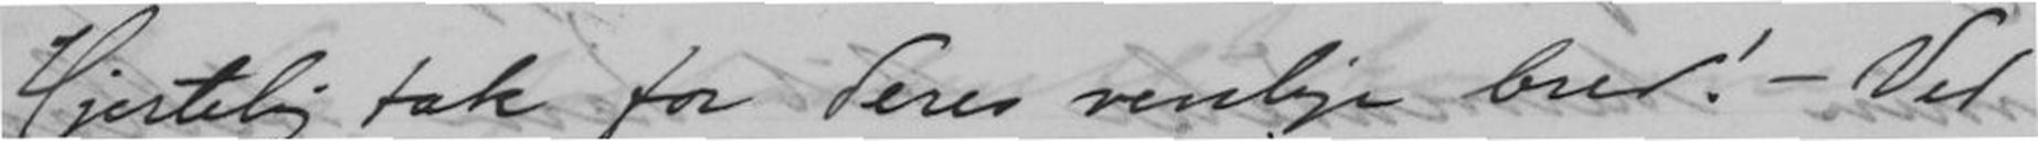Hjertelig tak for deres venlige brev! - Ved
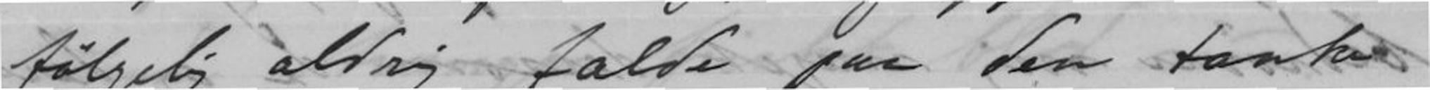følgelig aldrig falde paa den tanke
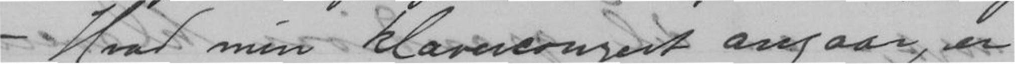- Hvad min klaverconzert angaar, er
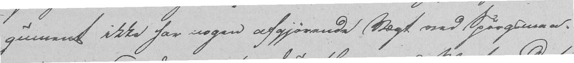gument ikke har nogen afgjørende Vægt ved Spørgsmaa-
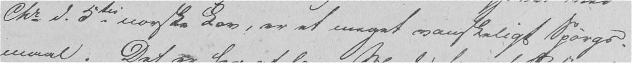Chr. d. 5tes norske Lov, er et meget vanskeligt Spørgs-
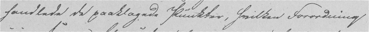handlede de paaklagede Punkter, hvilken Forordning
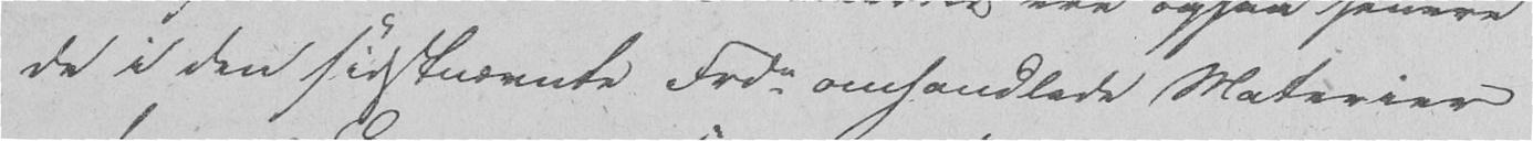de i den sidstnævnte Frdn omhandlede Materier
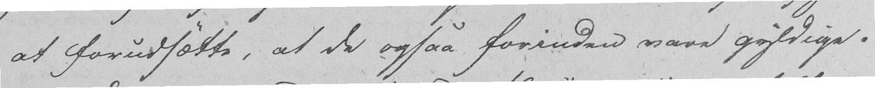at forudsætte, at de ogsaa forinden vare gyldige.
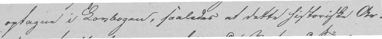optagne i Lovbogen, saaledes at dette historiske Ar-
